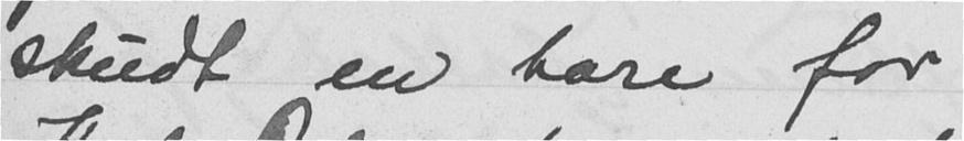skudt en hare for
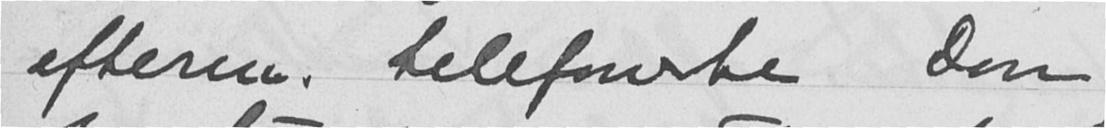efterm. telefonerte Don
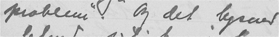problem". Og det lysned
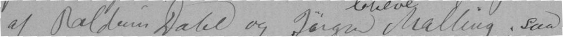af Balduin Dahl og Jørgen  Malling; saa
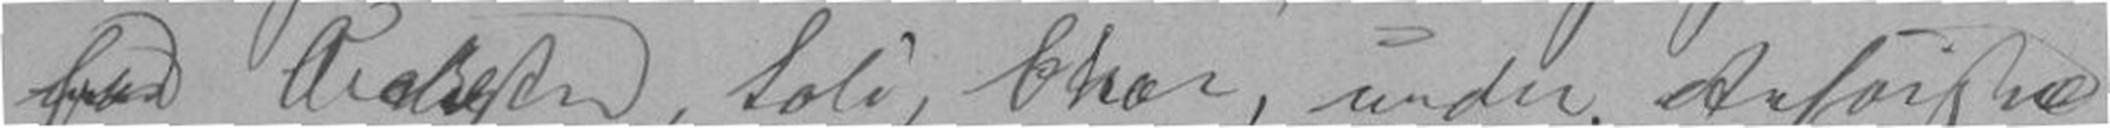for Orchester, Soli, Chor, under Anførske
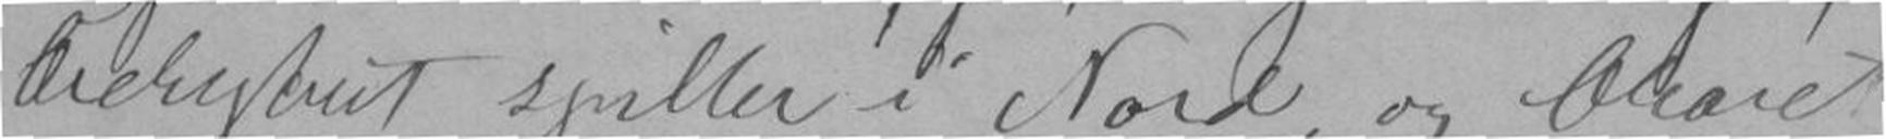Orchesteret spiller i Nord, og Choret
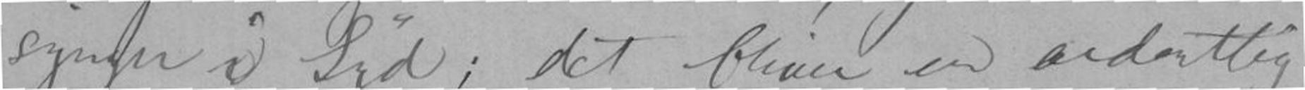synger i Syd; det bliver en ordentlig
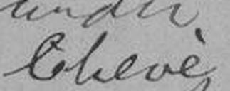Cheve
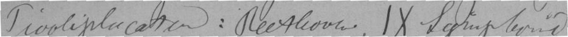Tivoliplacaten: Beethoven, IX Symphonie
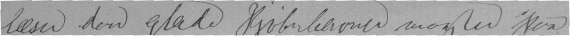læser den glade Kjøbenhavner maaske paa
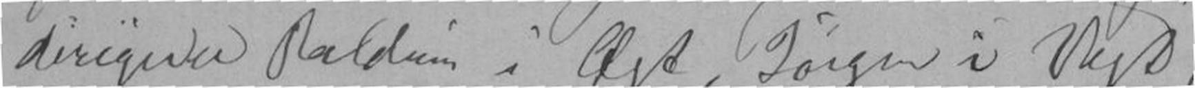dirigerte Balduin i Øst, Jørgen i Vest,
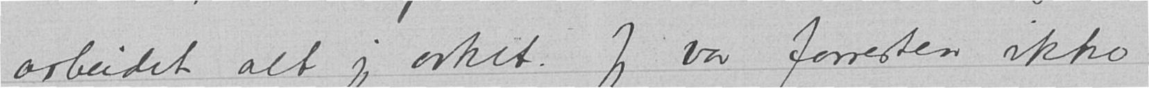arbeidet alt jeg orket. Jeg var forresten ikke
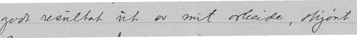godt resultat ut av mit arbeide, skjønt
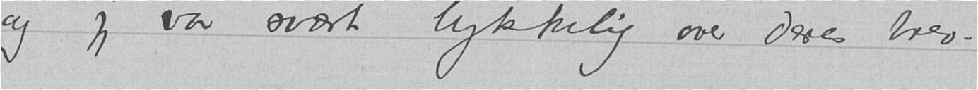og jeg var svært lykkelig over Deres brev.
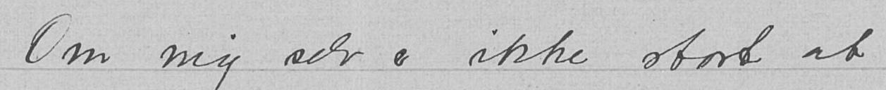Om mig selv er ikke stort at
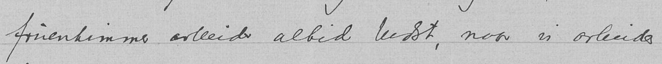fruentimmer arbeider altid bedst, naar vi arbeider
